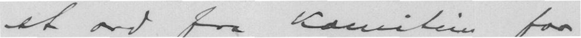et ord fra Komitéen for
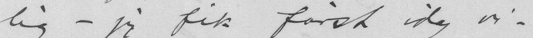lig - jeg fik først idag vi-
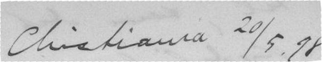Christiania 20/5.98
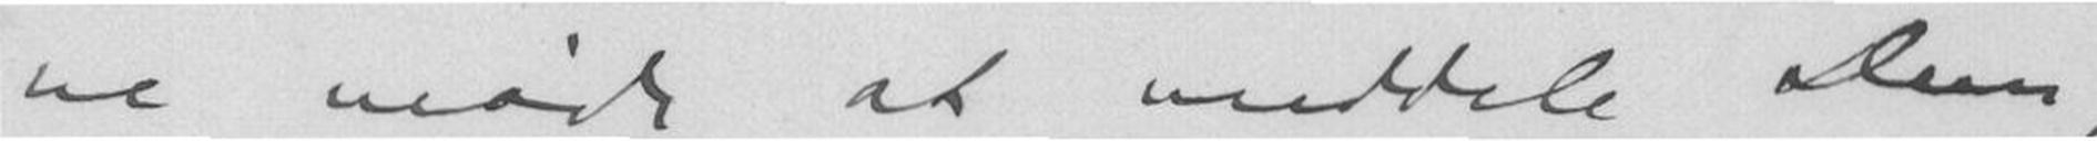ne måde at meddele Dem,
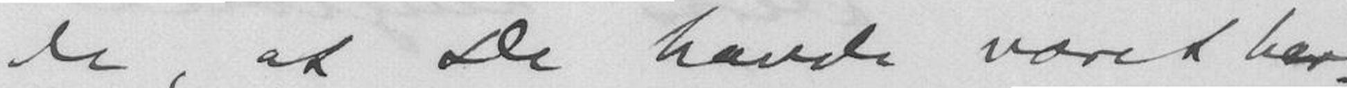de, at De havde været her
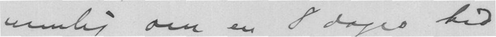nemlig om en 8 dages tid
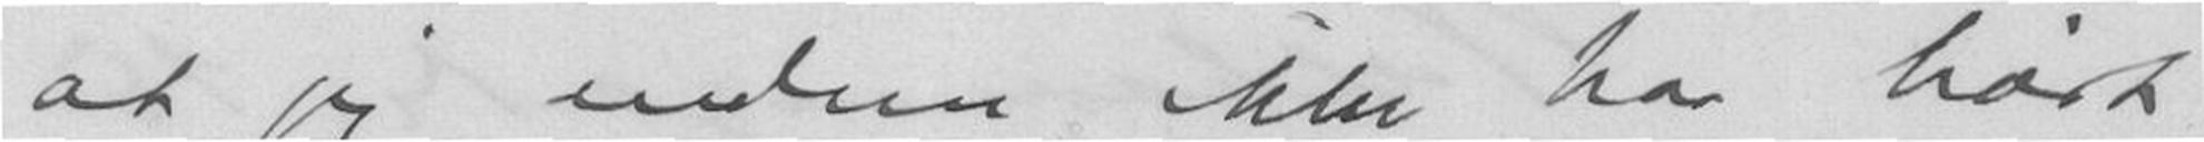at jeg endnu ikke har hørt
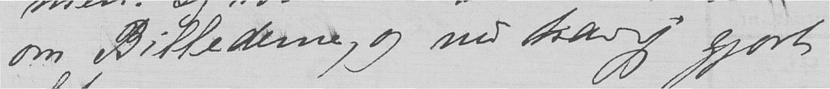om Billederne, og nu har jeg gjort
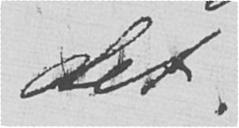det.
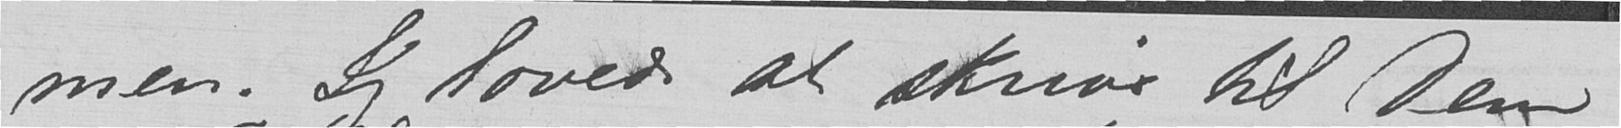men. Jeg lovede at skrive til Dem
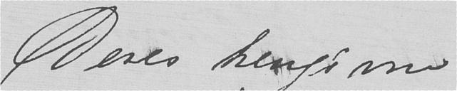Deres hengivne
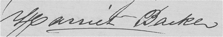Harriet Backer
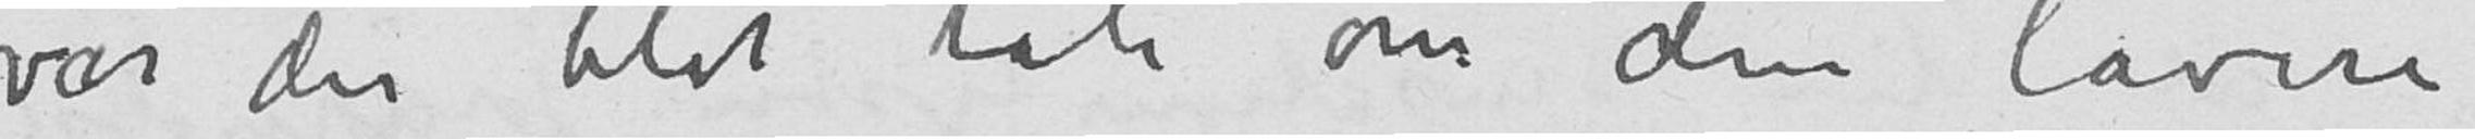var der blot tale om den lavere
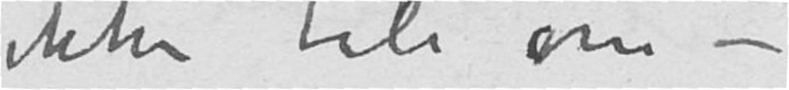ikke tale om –
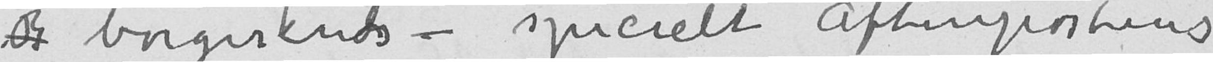B borgerkreds – specielt Aftenpostens
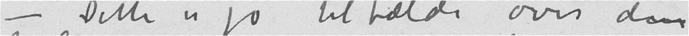– Dette er jo tilfælde over den
